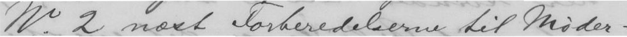N. 2 næst Forberedelserne til Møder-
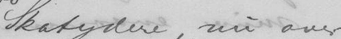Skatydere, nu over
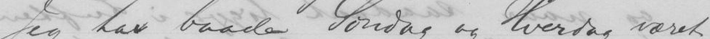Jeg har baade Søndag og Hverdag været
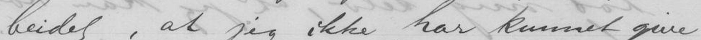beidet, at jeg ikke har kunnet give
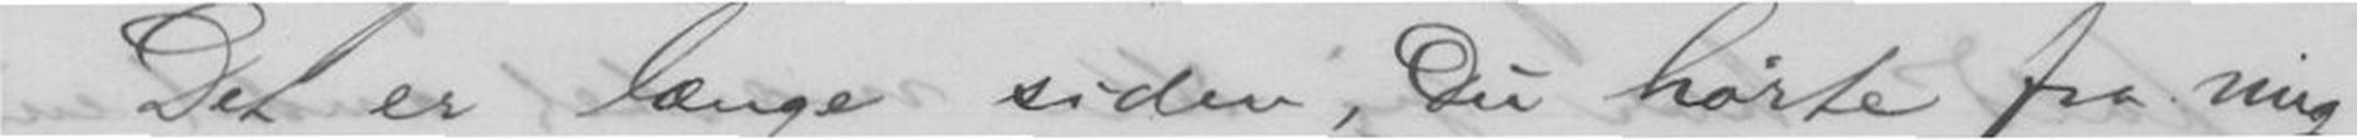Det er længe siden, Du hørte fra mig
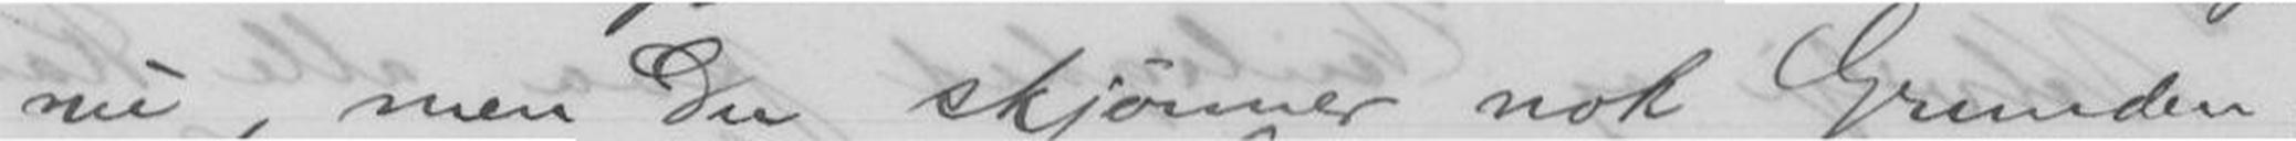nu, men Du skjønner nok Grunden.
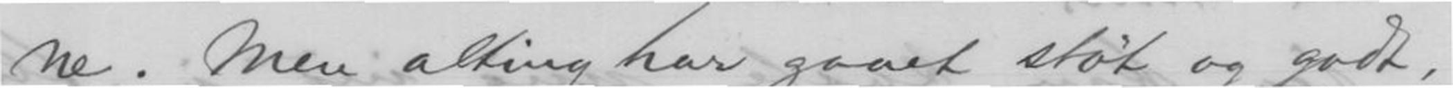ne. Men alting har gaaet støt og godt,
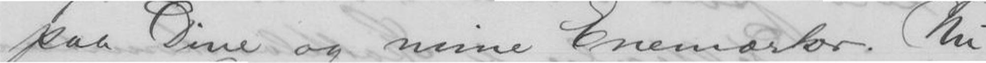paa Dine og mine Enemærker. Nu
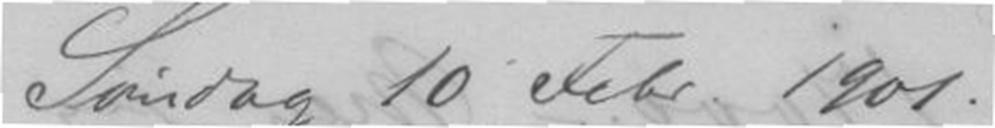Søndag 10 Febr 1901.
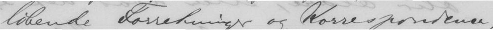løbende Forretninger og Korrespondence,
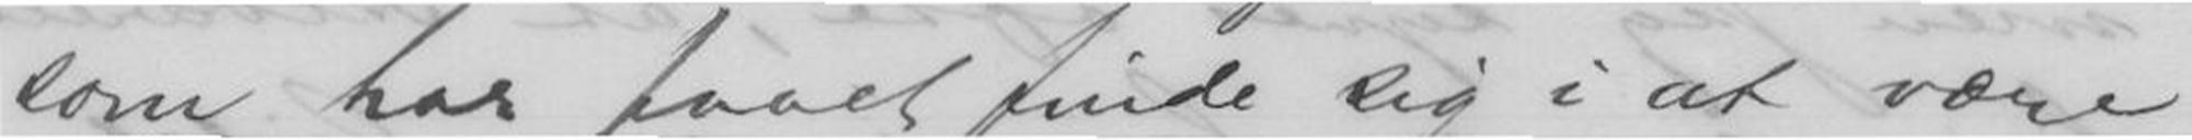som har faaet finde sig i at være
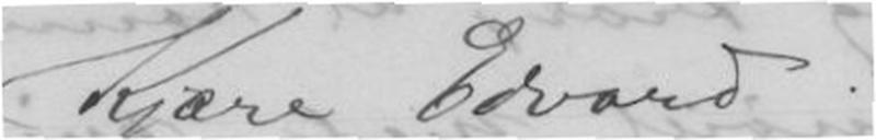Kjære Edvard!
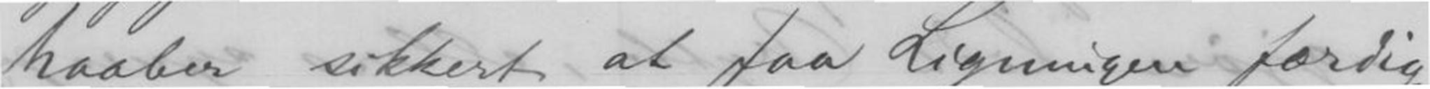haaber sikkert at faa Ligningen færdig
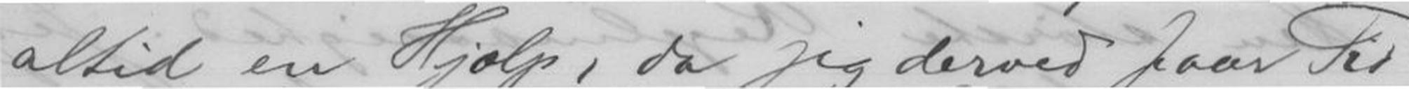altid en Hjælp, da jeg derved faar Tid
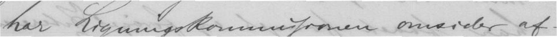har Ligningskommisionen omsider af-
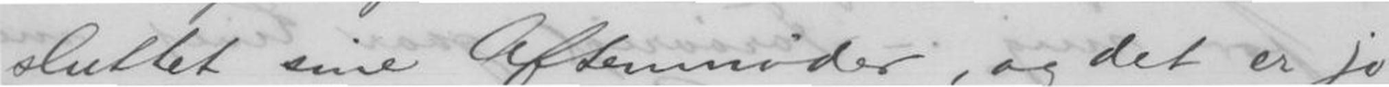sluttet sine Aftenmøder, og det er jo
